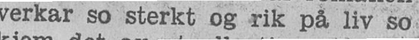verkar so sterkt og rik på liv so-
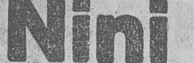Nini
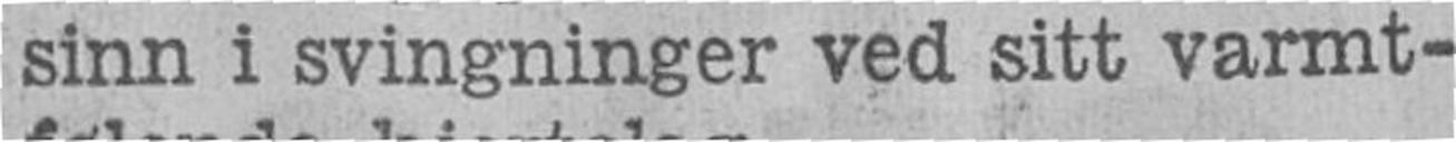sinn i svingninger ved sitt varmt-
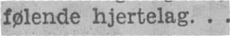følende hjertelag...
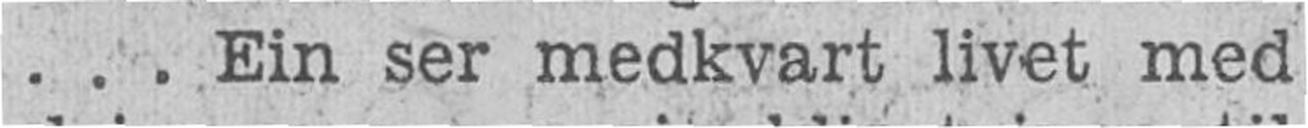... Ein ser medkvart livet med
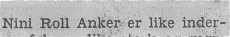Nini Roll Anker er like inder-
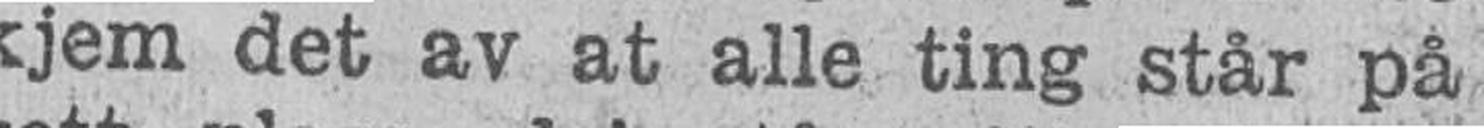kjem det av at alle ting står pâ
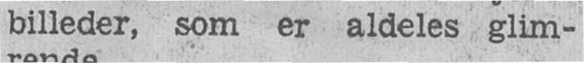billeder, som er aldeles glim-
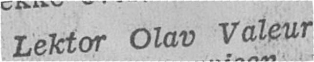Lektor Olav Valeur
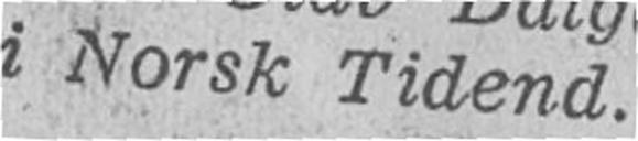i Norsk Tidend.
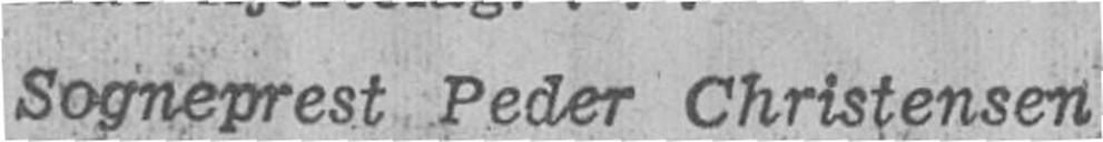Sogneprest Peder Christensen
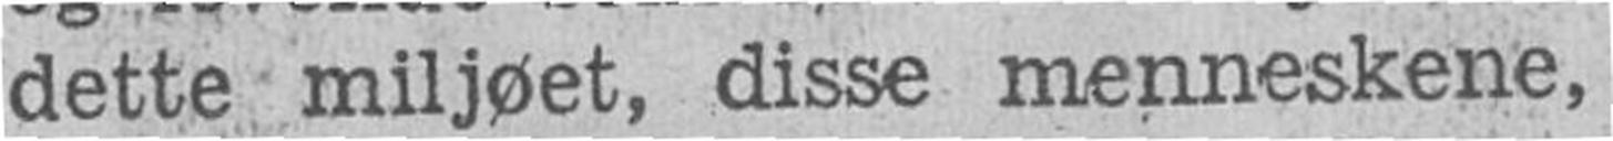dette miljøet, disse menneskene,
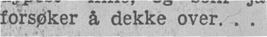forsøker å dekke over.
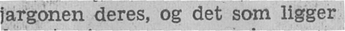jargonen deres, og det som ligger
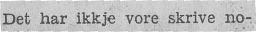Det har ikkje vore skrive no-
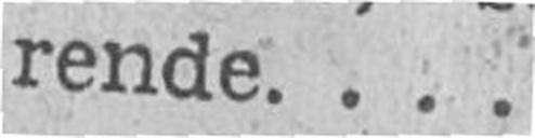rende....
